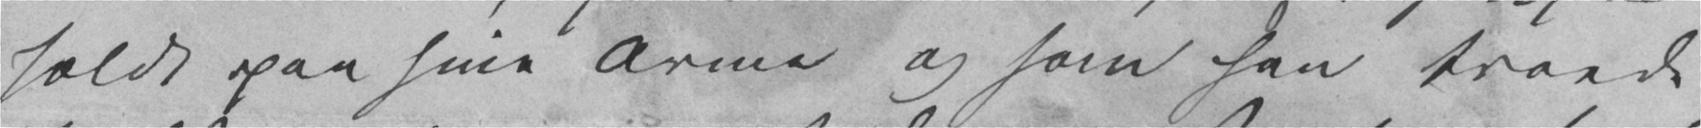holdt paa sine Arme og som han troede
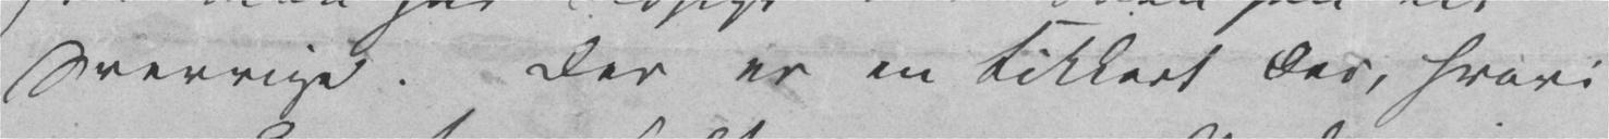Sverrige. Der er en Kikkert der, hvori
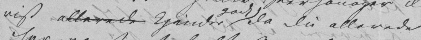vist allerede kjender godt da Du allerede
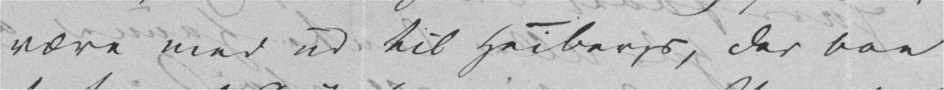være med ud til Heibergs, der boe
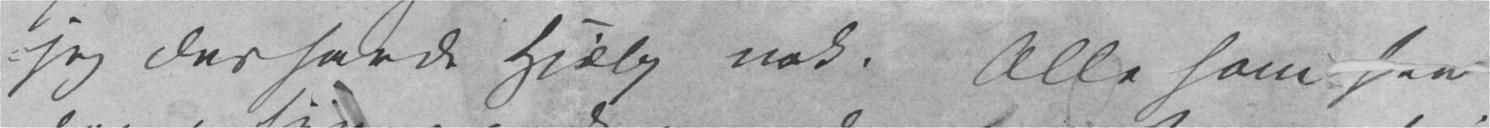jeg der havde Hjælp nok. Alle som see
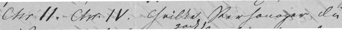Chr II, Chr. IV, hvilke Personager Du
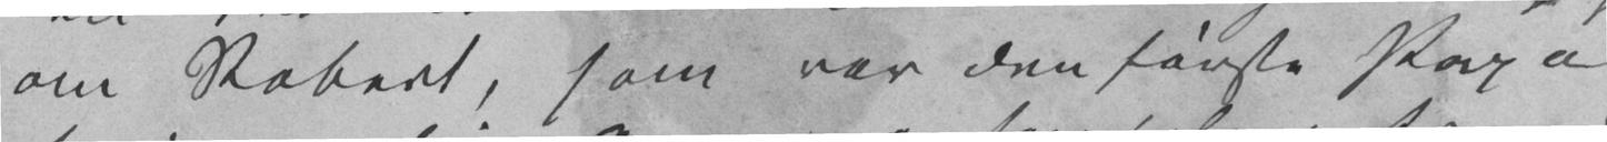om Robert, som var den første Papa
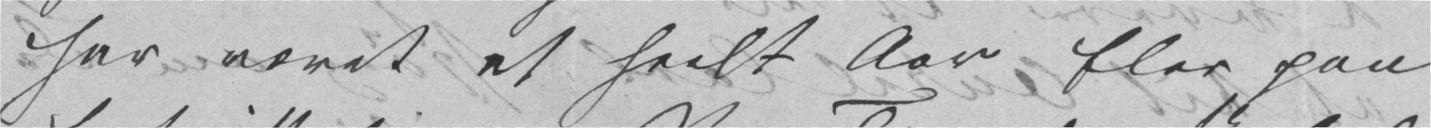har været et heelt Aar Elev paa
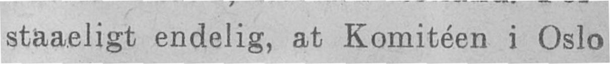staaeligt andelig, at Komiteen i Oslo
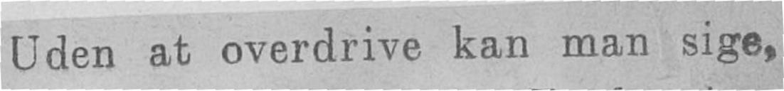Uden at overdrive kan man sige,
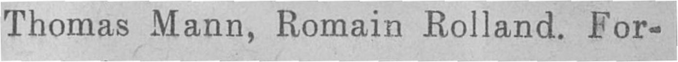Thomas Mann, Romain Rolland. For-
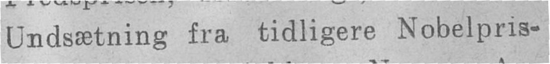Undsætning fra tidligere Nobelpris-
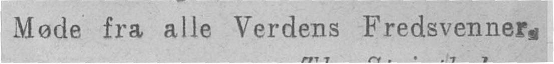Møde fra alle Verdens Fredsvenner.
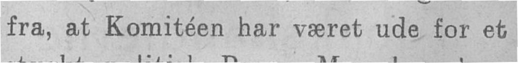fra, at Komiteen har været ude for et
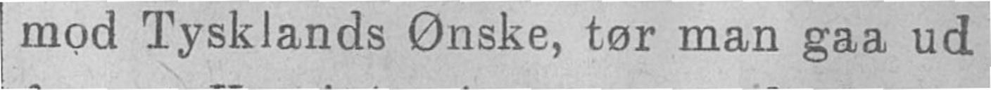mod Tysklands Ønske, tør man gaa ud
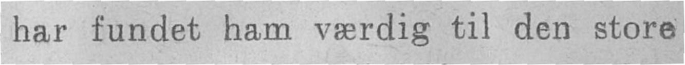har fundet ham værdig til den store
